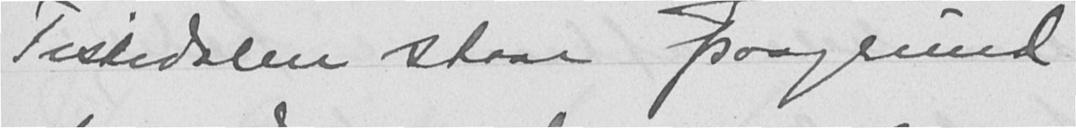Tistedalen staar paagrund
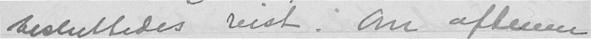besluttedes reist. Om aftenen
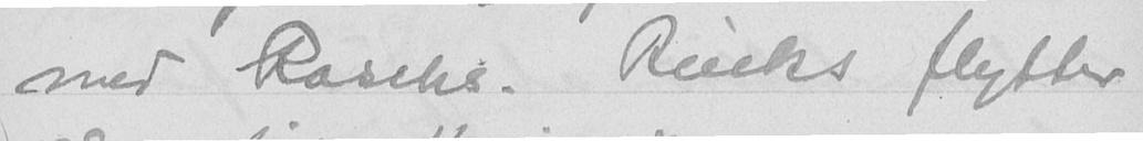med Raschs. Riecks flytter
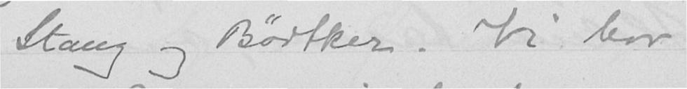Stang og Bødtker. Vi bor
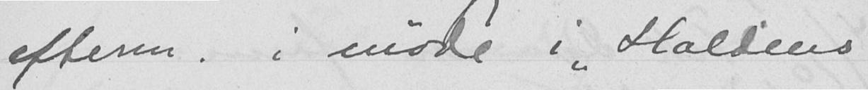efterm. i møde i "Haldens
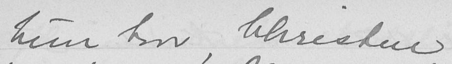hun tror, Christen
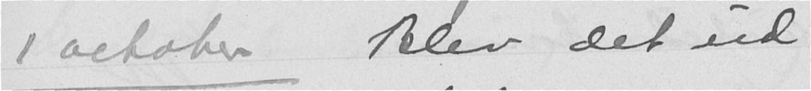1 october Blev det ud
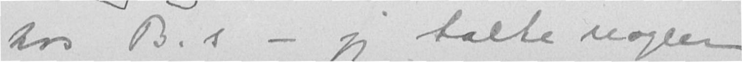hos B.s - jeg talte nogen
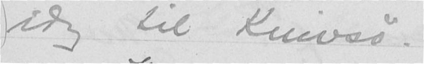idag til Knivsø.
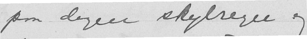paa dagen skylregn og
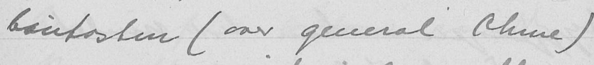bautasten (over general Ohme)
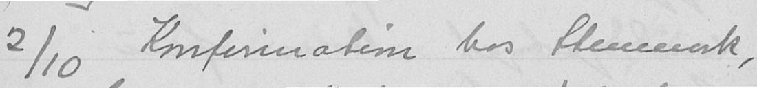2/10 Konfirmation hos Stenmark,
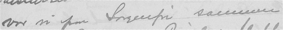var vi paa Sorgenfri sammen
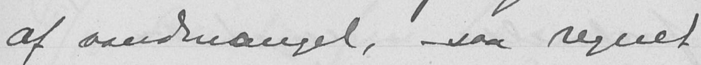af vandmangel, saa regnet
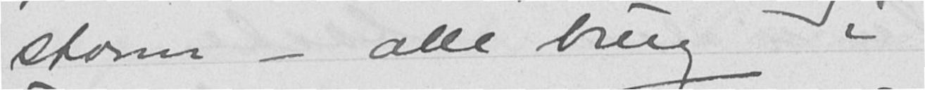storm - alle brug i
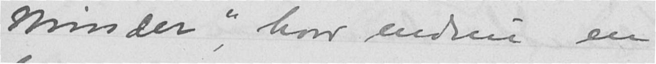Minder", hvor endnu en
